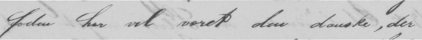foden har vel været den danske, der
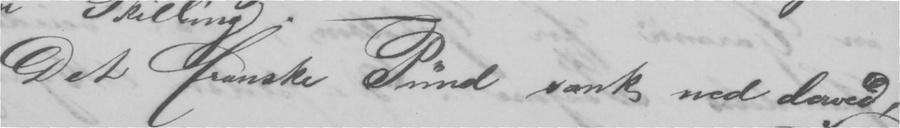Det Danske Pund sank med derved,
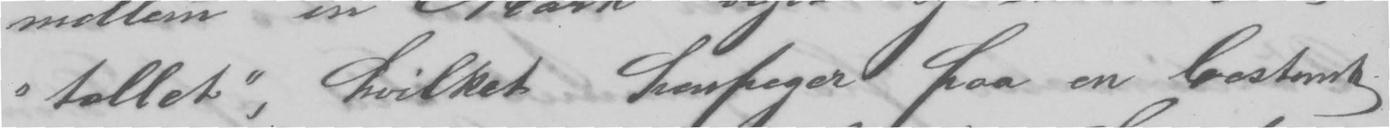"tællet", hvilket henpeger paa en bestemt
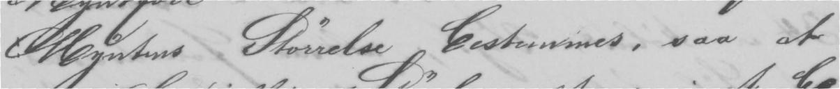Myntens Størrelse bestemmes, saa at
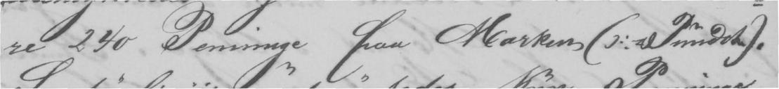re 240 Penninge paa Marken (ɔ: Pundet).
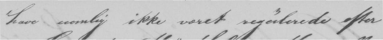have nemlig ikke været regulerede efter
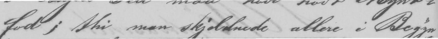fod; thi man skjelnede allere i Begyn-
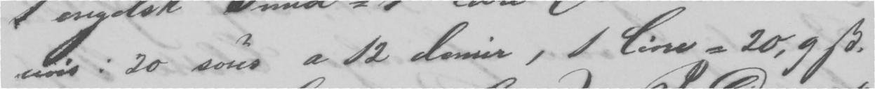nois: 20 sous a 12 demir, 1 livre = 20,9 ẞ.
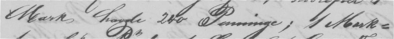Mark havde 240 Penninge; 1 Mark =
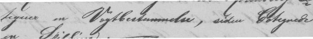tegner en Vægtbestemmelse, siden betegnede
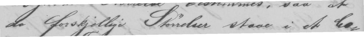de forskjellige Størrelser staae i et be-
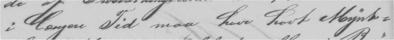i langen Tid maa heve hvert Mynt-
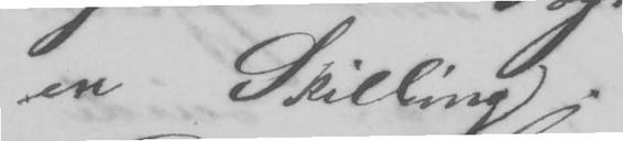en Skilling.
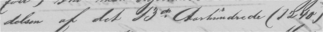delsen af det 13de Aarhundrede (1240)
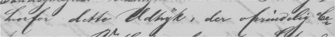hvorfor dette Udtryk, der opindelig be-
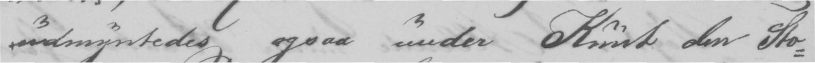udmyntedes ogsaa under Kvint den Sto-
